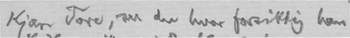Kjære Tore, ser du hvor forsiktig han
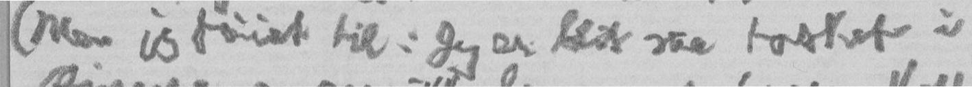(Men jeg føiet til: Jeg er blit saa tosket i
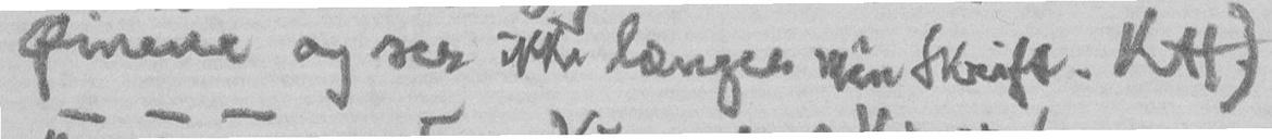Øinene og ser ikke længer min Skrift. KH.)
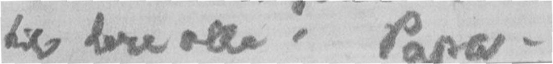til dere alle. Papa.
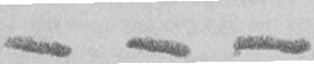- - -
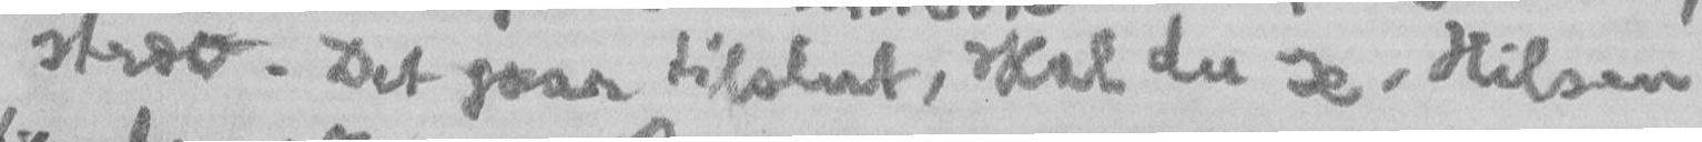stræv. Det gaar tilslut, skal du se. Hilsen
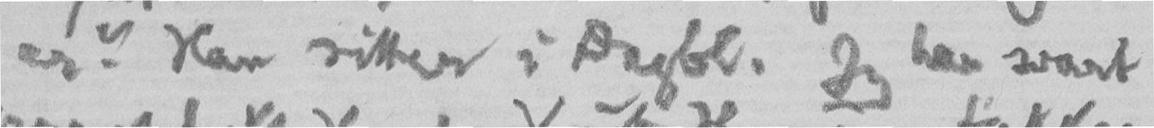er? Han sitter i Dagbl. Jeg har svart
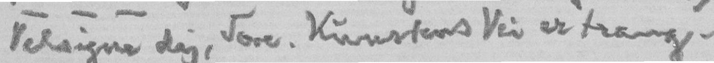Velsigne dig, Tore. Kunstens Vei er trang.
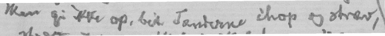Men gi ikke op, bit Tænderne ihop og stræv,
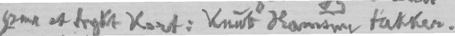paa et trykt Kort: Knut Hamsun takker.
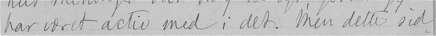har været activ med i det. Men dette sid-
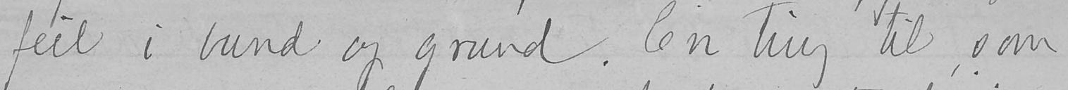feil i bund og grund. En ting til, som
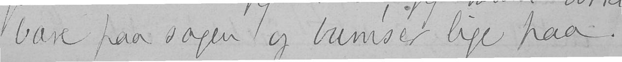bare paa sagen og bumser lige paa.
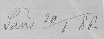Paris 20/1 88.
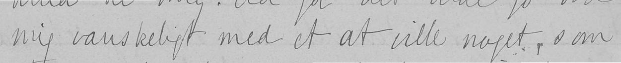mig vanskeligt med et at ville noget, som
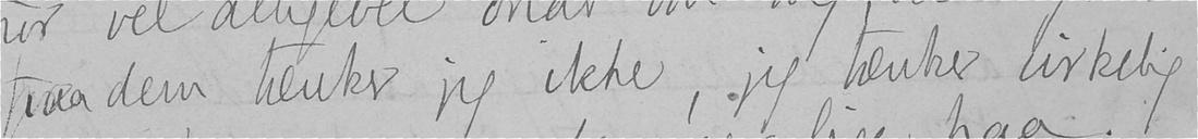paa dem tænker jeg ikke, jeg tænker virkelig
